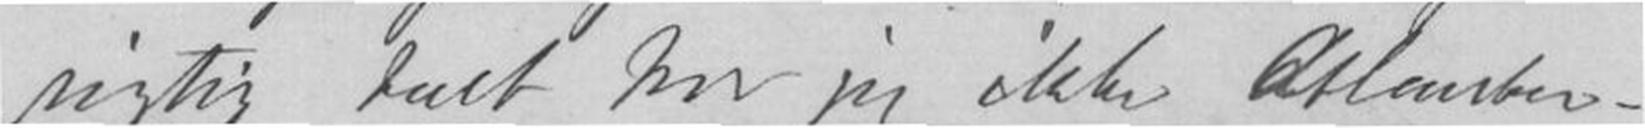rigtig talt har jeg ikke
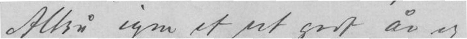Altså igen et ut godt år er
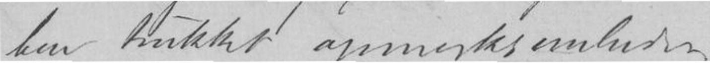har trukket opmerksomheden
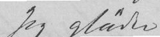Jeg glæder
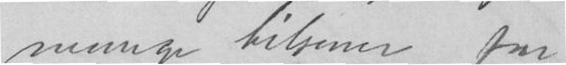mange hilsner fra
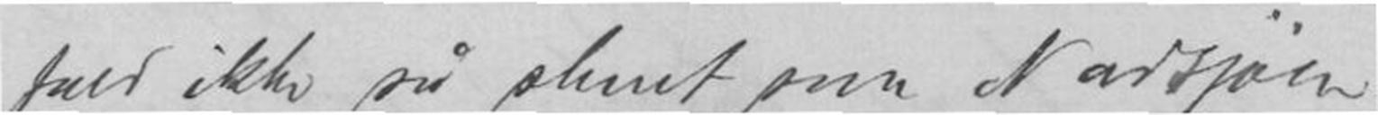fald ikke så skent som Nordsjøen
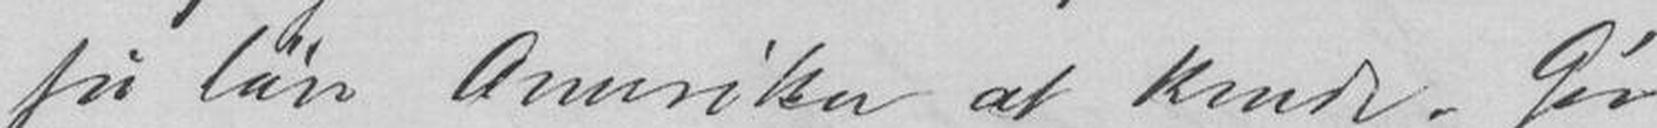få lære Amerika at kende. Giv
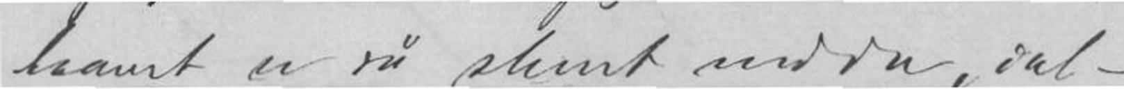er så skent , ial-
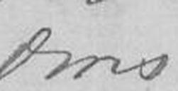Deres
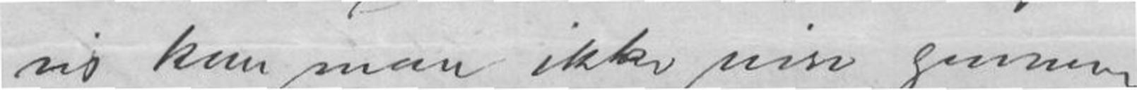vis kan man ikke reise gennem
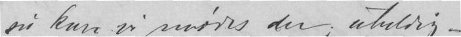så kan vi mødes der, uheldig-
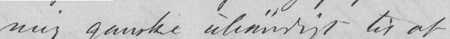mig ganske til at
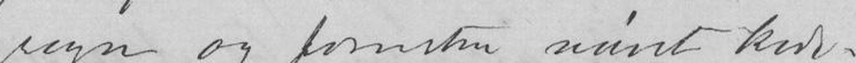regn og vært kede-
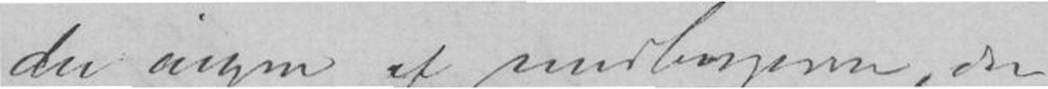der ingen af medborgerne, der
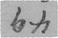49
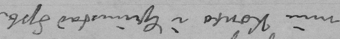min Konto i Grimstad Spb.
